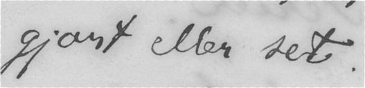gjort eller set.
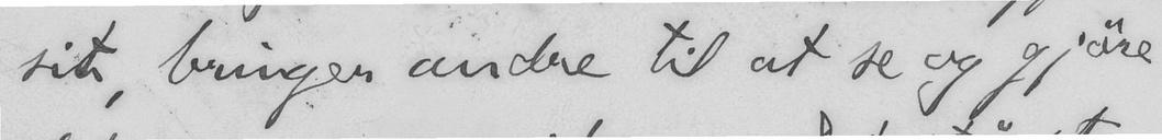sit, bringer andre til at se og gjøre
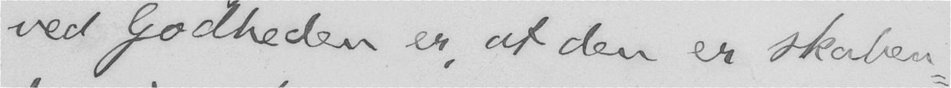ved Godheden er, at den er skaben-
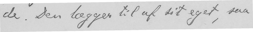de. Den lægger til af sit eget, saa
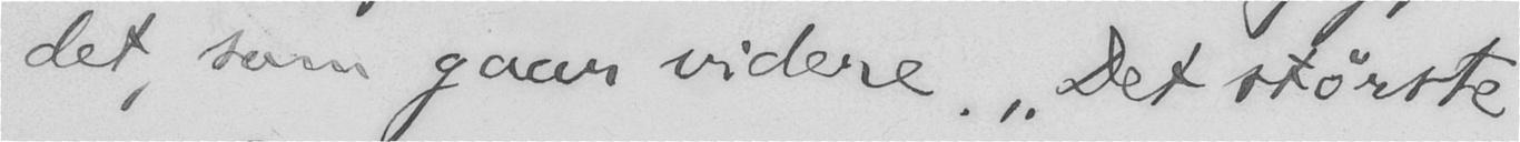det, som gaar videre. "Det største
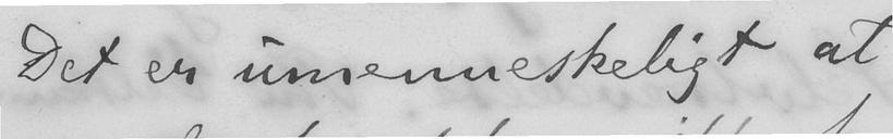Det er umenneskeligt at
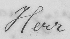Herr
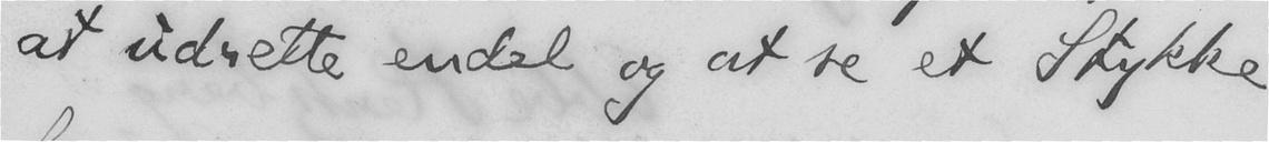at udrette endel og at se et Stykke
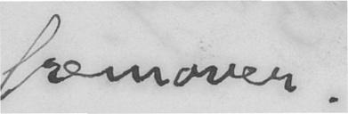fremover.
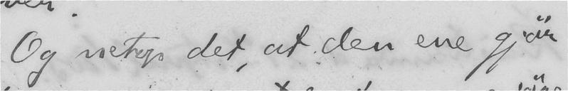Og netop det, at den ene gjør
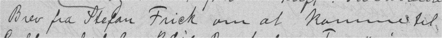Brev fra Stefan Frick om at komme til
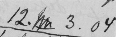12. 3.04
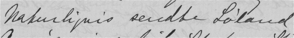Naturligvis sendte Løland
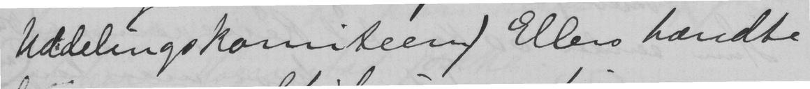Uddelingskomiteen) Ellers hændte
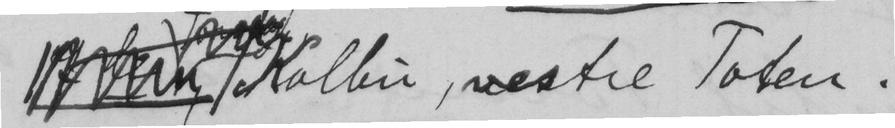Kolbu, vestre Toten.
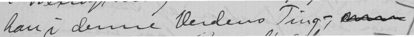han i denne verdens Ting;
